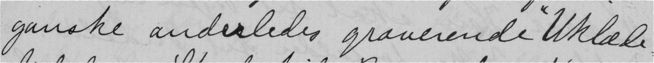ganske anderledes graverende "Uklæde-
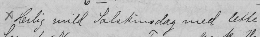x Herlig mild Solskinsdag med lette
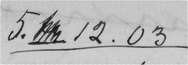5.  12.03
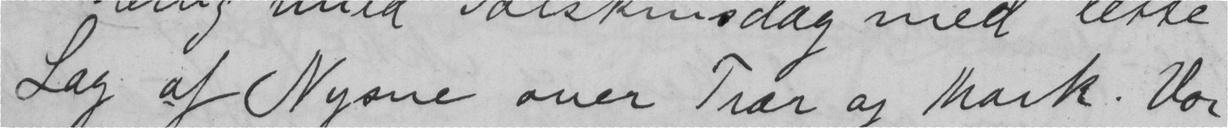Lag af Nysne over Trær og Mark. Vor
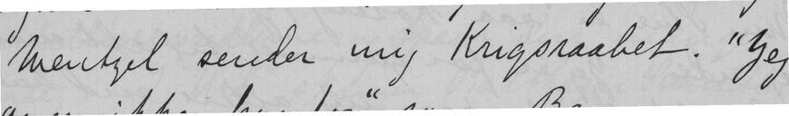Wentzel sender mig Krigsraabet. "Jeg
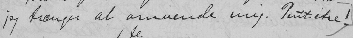jeg trænger at omvende mig. Peut etre!
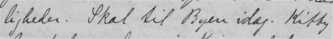ligheder. Skal til Byen idag. Kitty
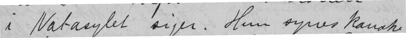i Natasylet siger. Hun synes kanske
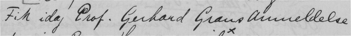Fik idag Prof. Gerhard Grans anmeldelse
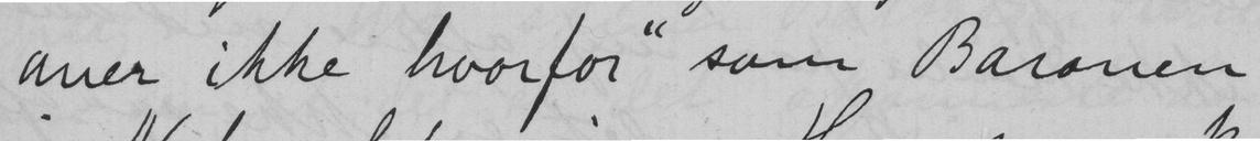aver ikke hvorfor" som Baronen
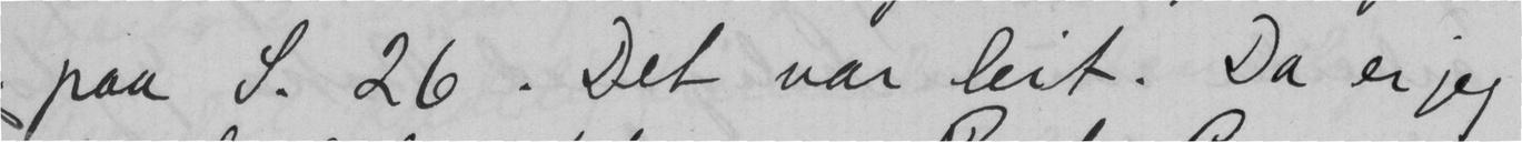paa S. 26. Det var leit. Da er jeg
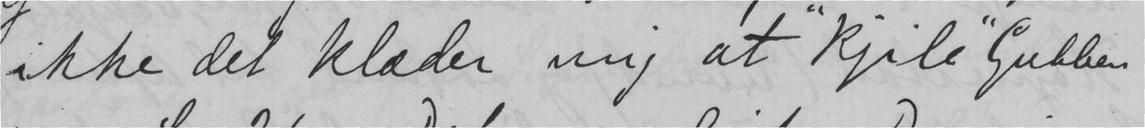ikke det klæder mig at "kjile" Gubben
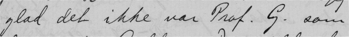glad det ikke var Prof. G. som
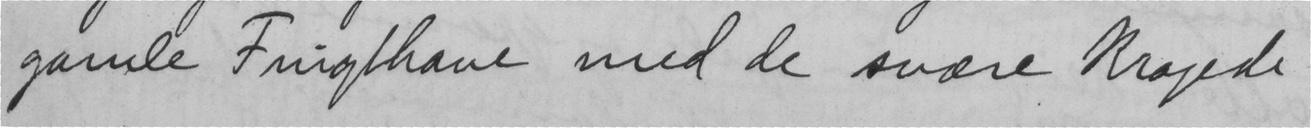gamle Frugthave med de svære Krogede
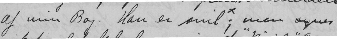af min Bog. Han er snil; men synes
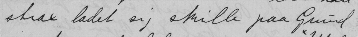strax ladet sig skille paa Grund
